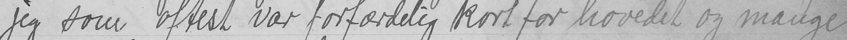jeg som oftest var forfærdelig kort for hovedet og mange
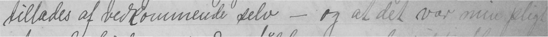tillades af vedkommende selv - og at det var min pligt
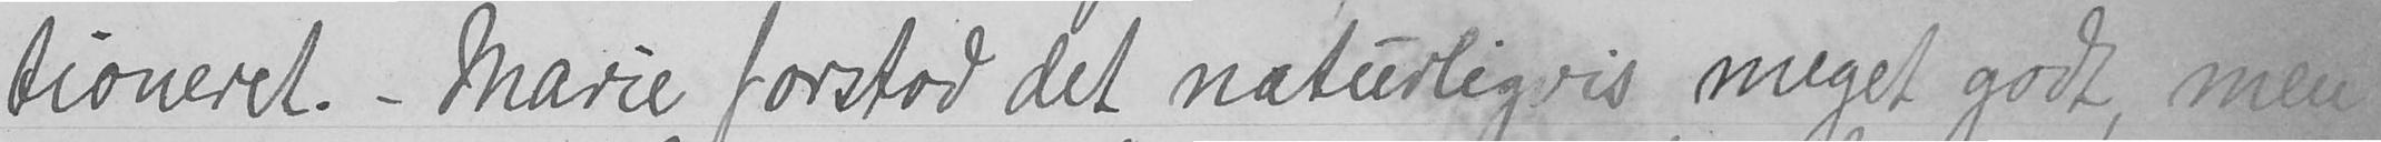tioneret. - Marie forstod det naturligvis meget godt, men
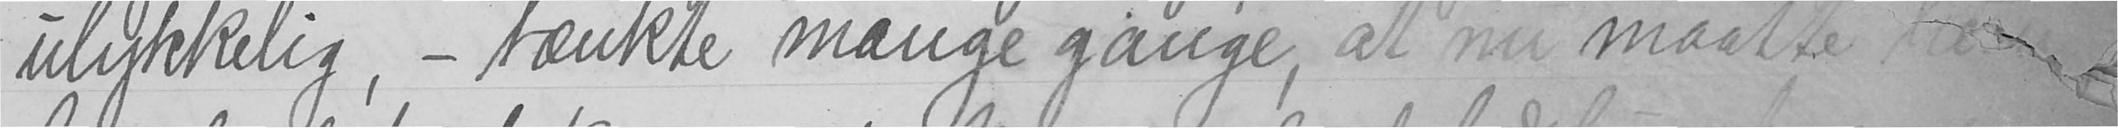ulykkelig, - tænkte mange gange, at nu maatte hun
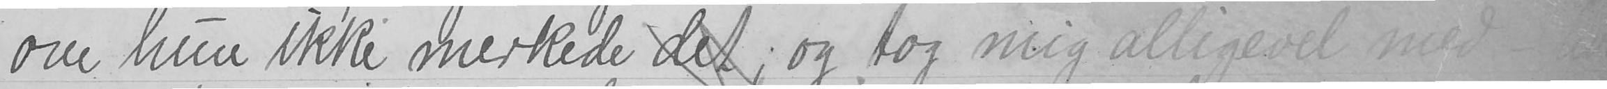om hun ikke merkede det; og tog mig alligevel med
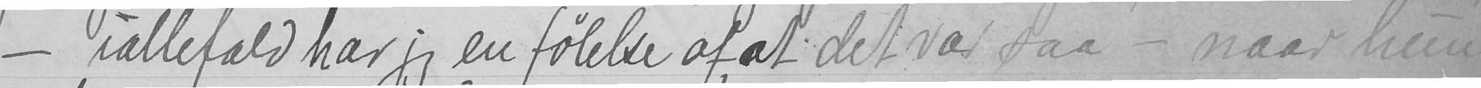- iallefald har jeg en følelse af at det var saa - naar hun
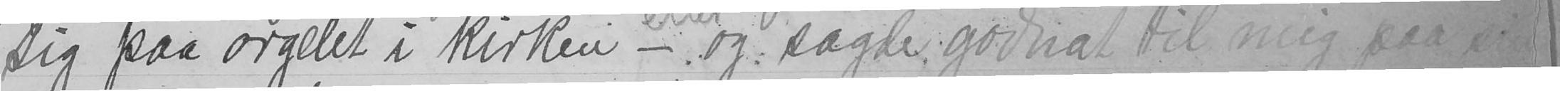sig paa orgelet i kirken - eller og sagde godnat til mig paa sin
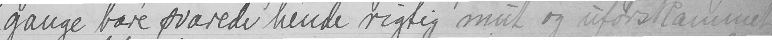gange bare svarede hende rigtig mut og uforskammet,
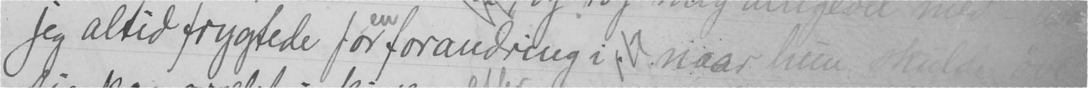jeg altid frygtede for en forandring i  naar hun skulde øve
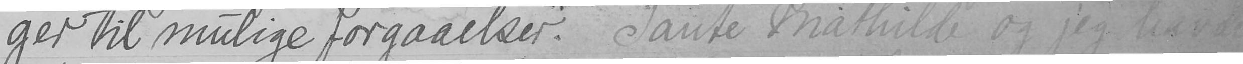ger til mulige forgaaelser. Tante Mathilde og jeg havde
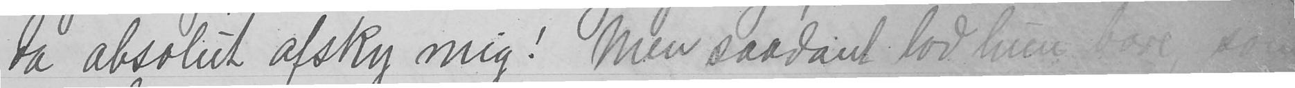da absolut afsky mig! Men saadant lod hun bare som
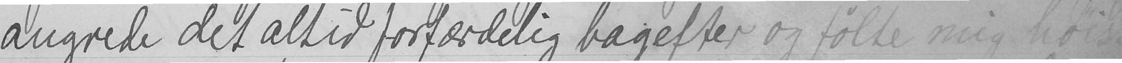angrede det altid forfærdelig bagefter og følte mit høist
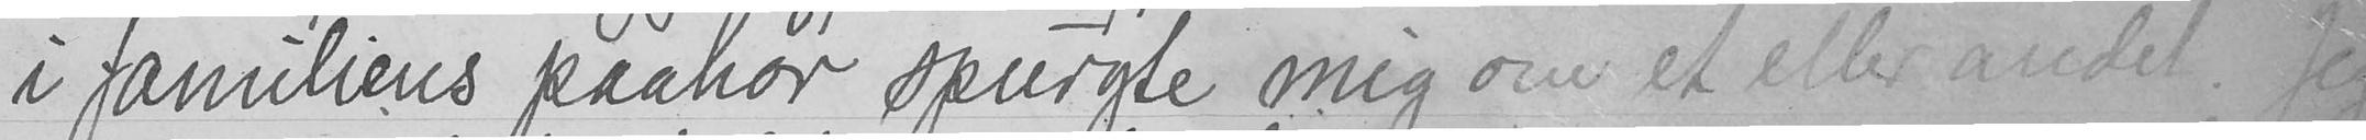i famliens paahør spurgte mig om et eller andet. Jeg
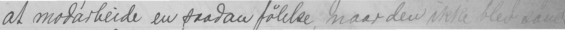at modarbeide en saadan følelse, naar den ikke blev san
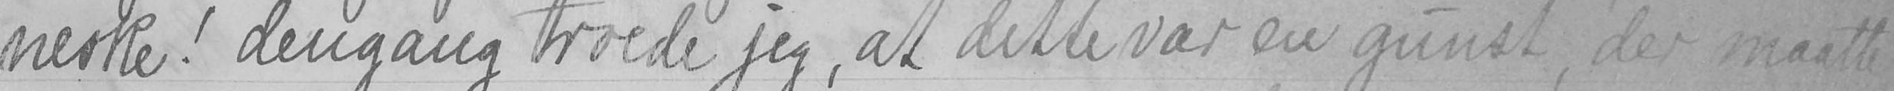neske! dengang troede jeg, at dette var en gunst, der maatte
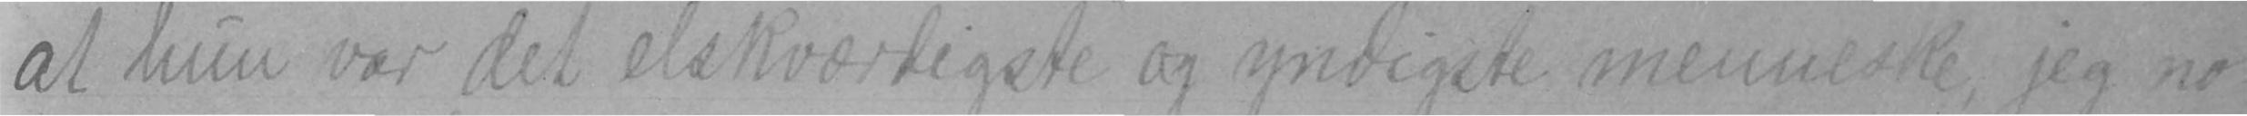at hun var det elskværdigste og yndigste menneske, jeg no-
